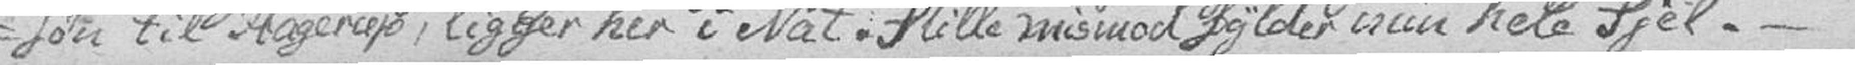-søn til Hagerup, ligger her i Nat. Stille mismod fylder min hele Sjel. –
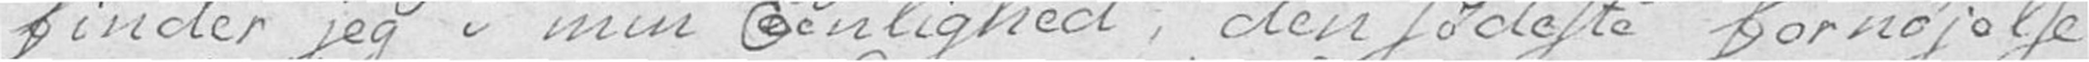finder jeg i min Eenlighed, den sødeste fornøjelse
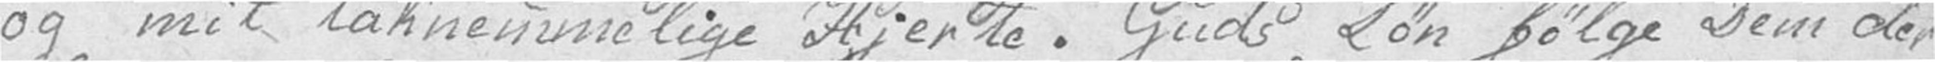og mit taknemmelige Hjerte. Guds Løn følge Dem der
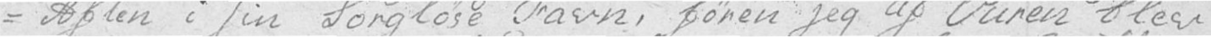-Aften i sin Sorgløse Favn, føren jeg af Luren blev
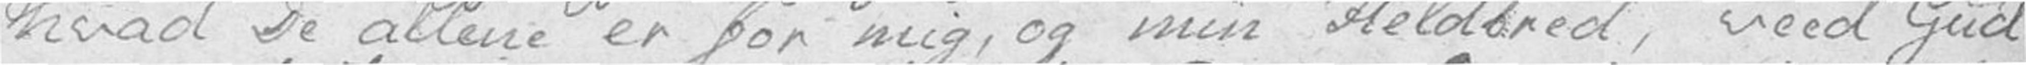hvad De allene er for mig, og min Heldbred, veed Gud
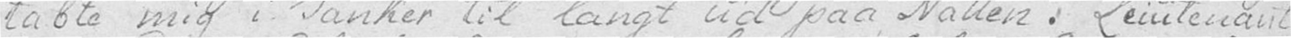tabte mig i Tanker til langt ud paa Natten. Leiutenant
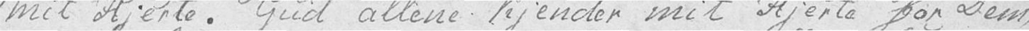mit Hjerte. Gud allene kjender mit Hjerte for Dem,
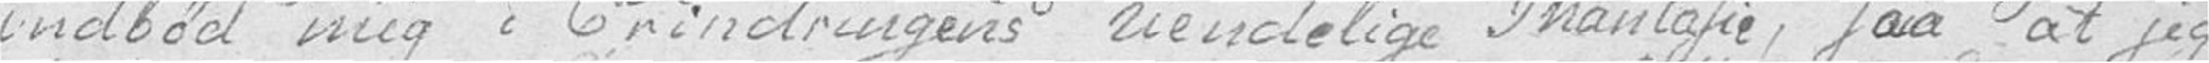indbød mig i Erindringens uendelige Phantasie, saa at jeg
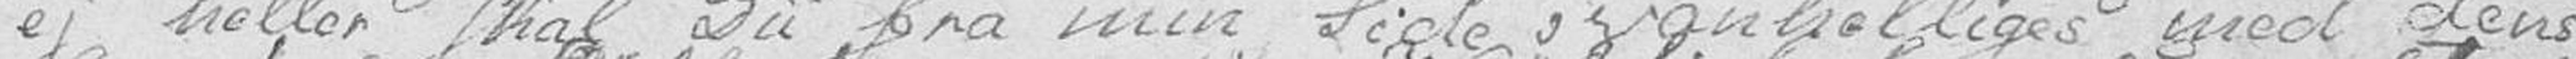ej heller skal Du fra min Side vanhelliges med dens
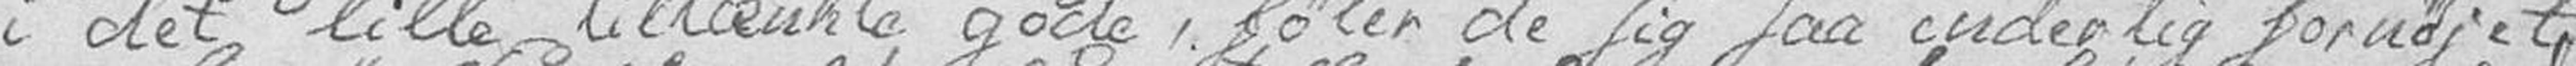i det lille tiltænkte gode, føler de sig saa inderlig fornøjet,
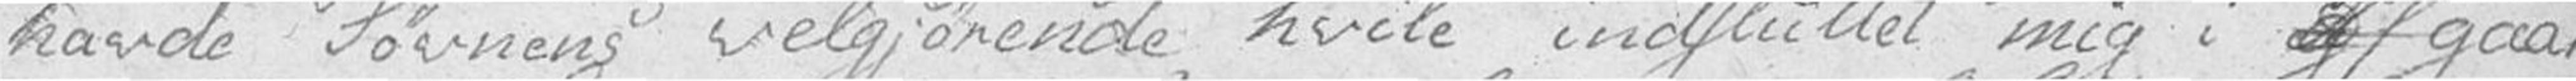havde Søvnens velgjørende hvile indsluttet mig i Af gaar
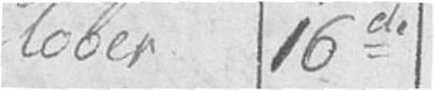tober 16de
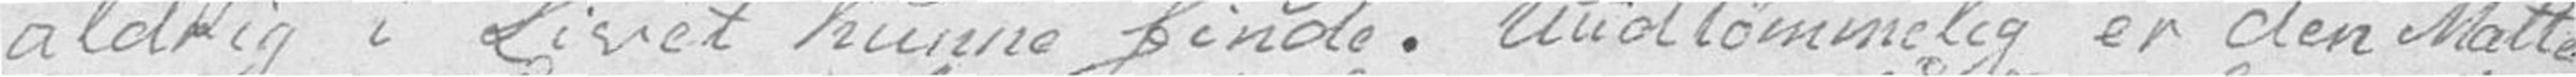aldrig i Livet kunne finde. Uudtømmelig er den Matte
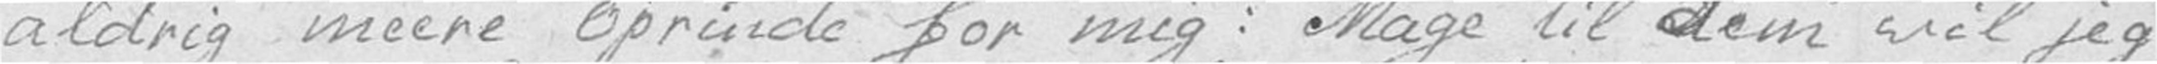aldrig meere oprinde for mig: Mage til dem vil jeg
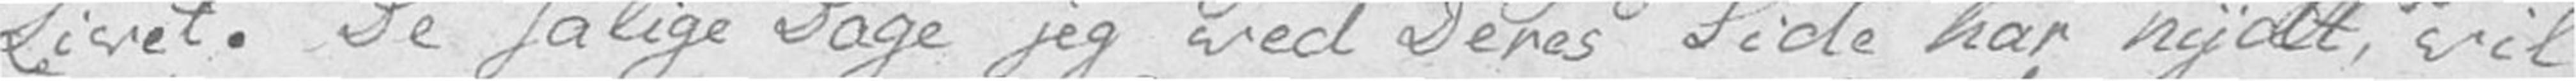Livet. De salige Dage jeg ved Deres Side har nydt, vil
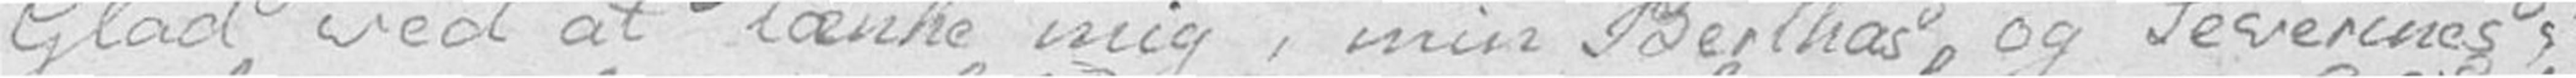Glad ved at tænke mig, min Berthas, og Severines
